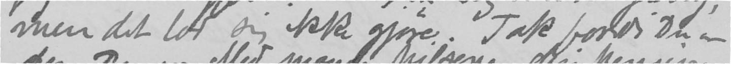men det lod sig ikke gjøre. Tak frordi Du er
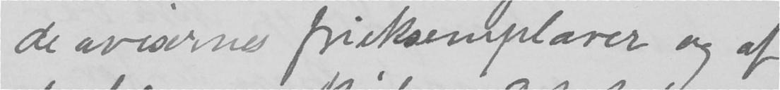de avisernes frieksemplarer og af
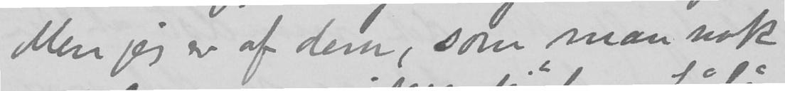Men jeg er af dem, som man nok
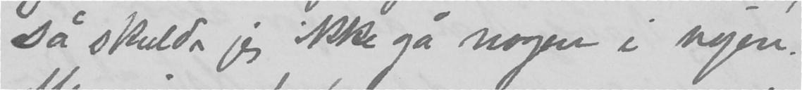så skulde jeg ikke gå nogen i vejen.
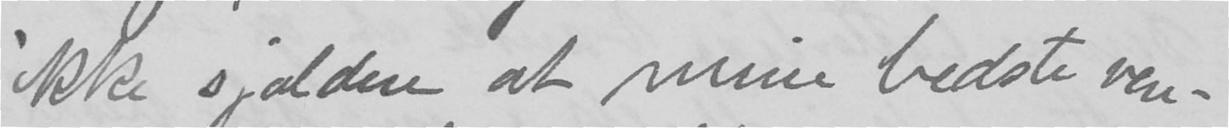ikke sjelden at mine bedste ven-
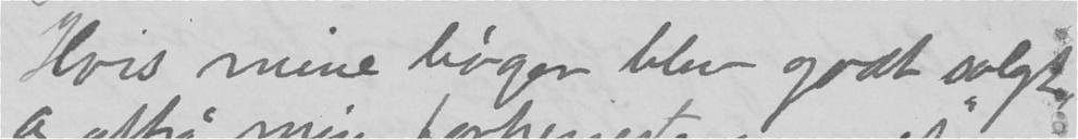Hvis mine bøger blev godt solgt,
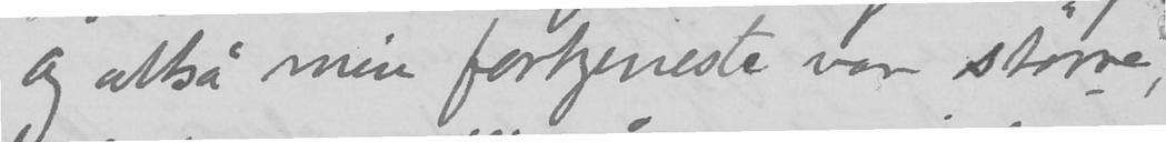og altså min fortjeneste var større
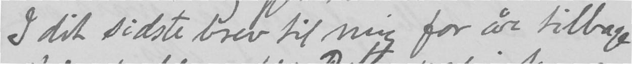I dit sidste brev til mig for år tilbage
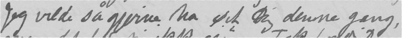Jeg vilde så gjerne ha set Dig denne gang,
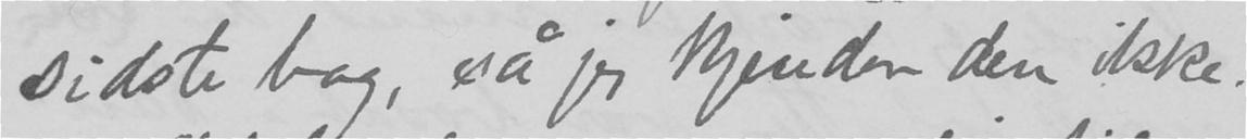sidste bog, sa jeg kjender den ikke.
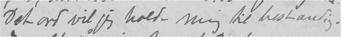Det ord vil jeg holde mig til bestandig.
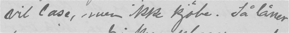vil læse, men ikke kjøbe. Så låner
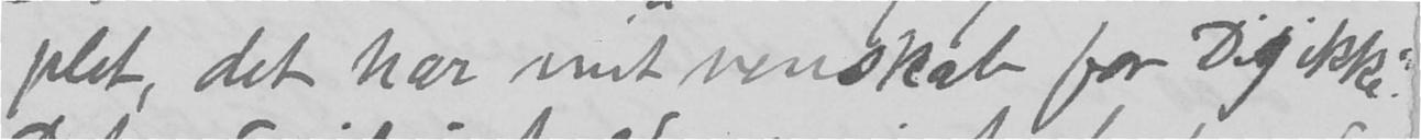plet, det har mit venskab for Dig ikke".
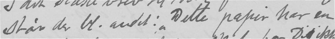står der bl. andet: "Dette papir har en
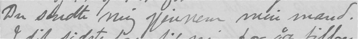Du sendte mig gjennem min mand.
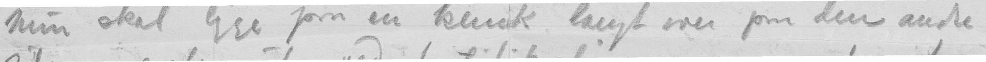hun skal ligge paa en klinik langt over paa den andre
